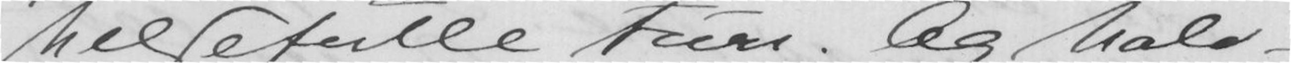helsefulle turer. Og halv-
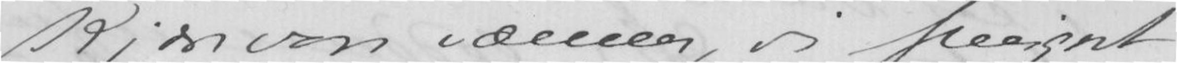Kjære vore vænner, vi smigret
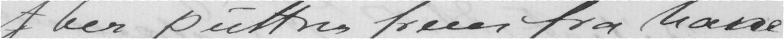f her puttrer frem fra hane
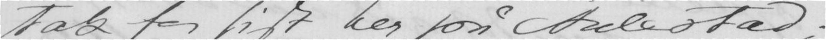tak for sist her på Aulestad;
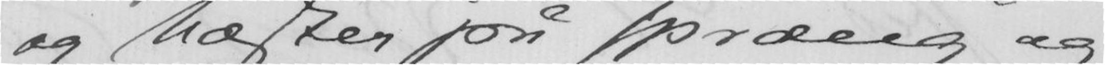og hæster på spræng og
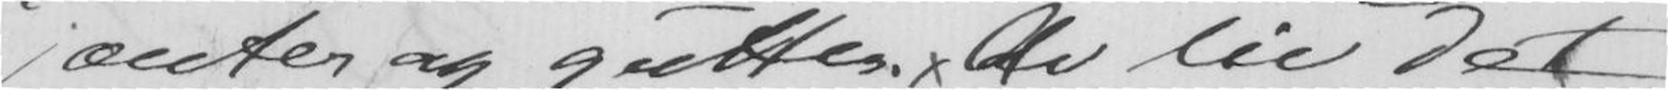jænter og gutter. xAv liv det
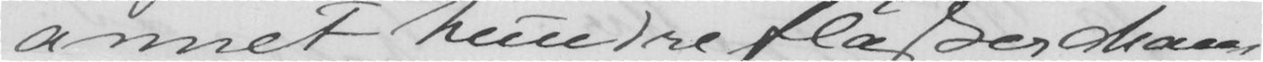annet hundre flasker cham-
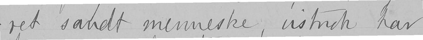ret sandt menneske, vistnok har
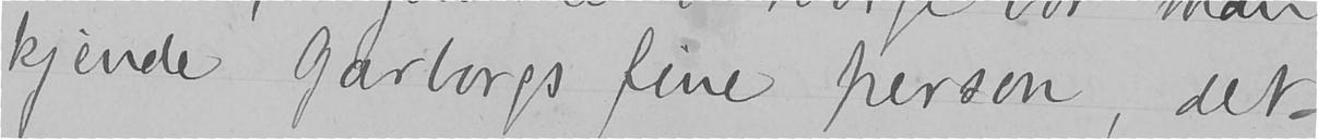kjende Garborgs fine person, det-
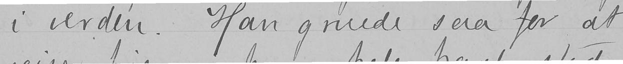i verden. Han gruede saa for at
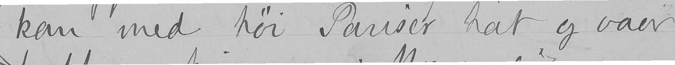kan med høi Pariser hat og vaar
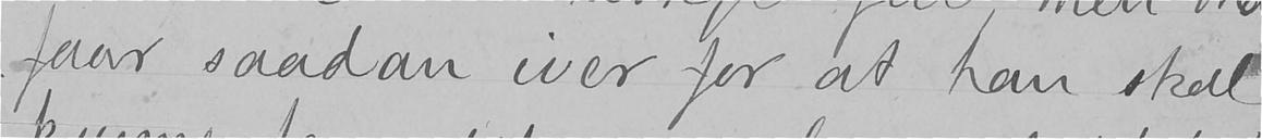faar saadan iver for at han skal
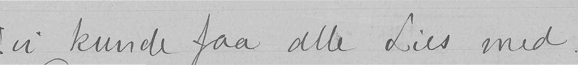vi kunde faa alle Lies med.
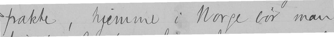frakk, hjemme i Norge bør man
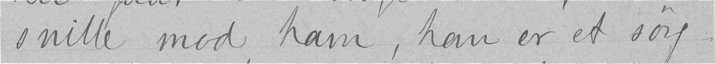snille mod ham, han er et sørg-
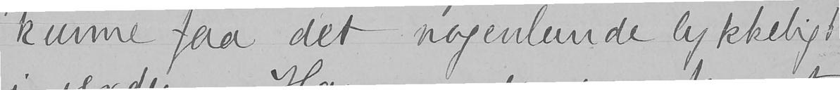kunne faa det nogenlunde lykkeligt
